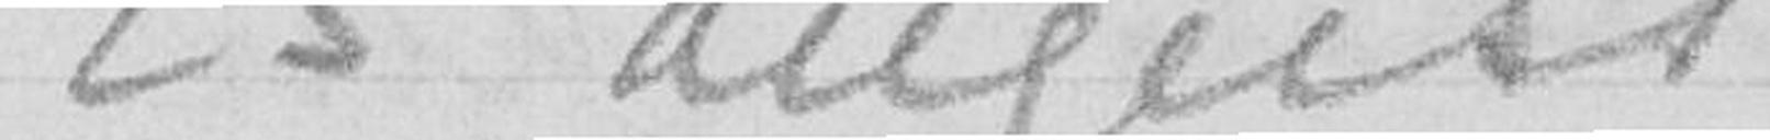25 august
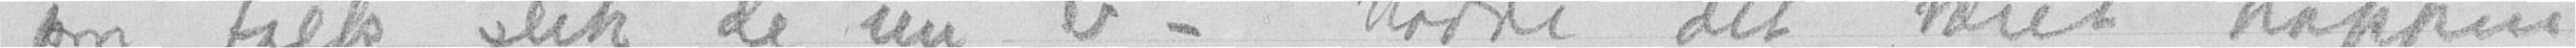paa folk, slik de nu er - hadde det været trappen
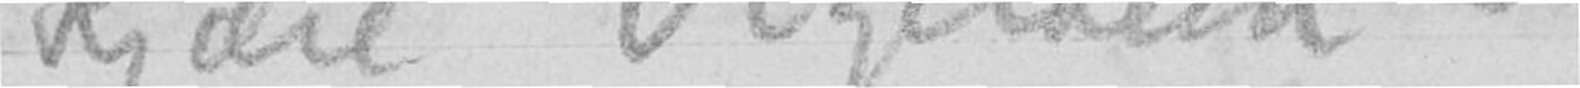Kjære Vigeland
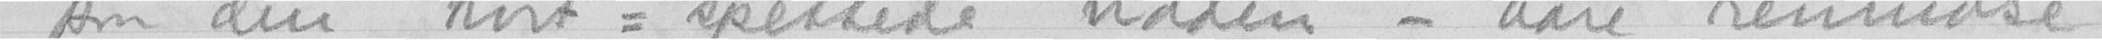paa den hvit-spettede vidden - bere renmose og
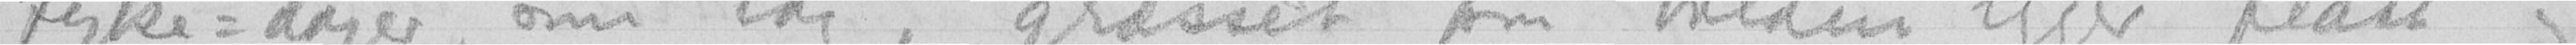fyke-dager, som idag, græsset paa volden ligger flatt og
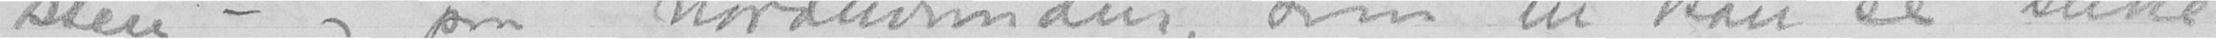sten - og paa nordenvinden, som en kan se slike
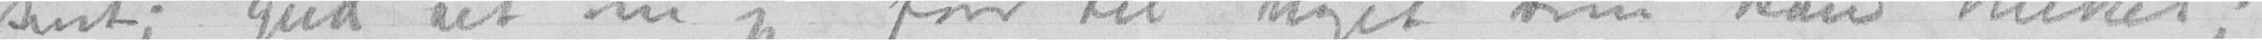sint; gud vet om jeg faar til noget som kan brukes;
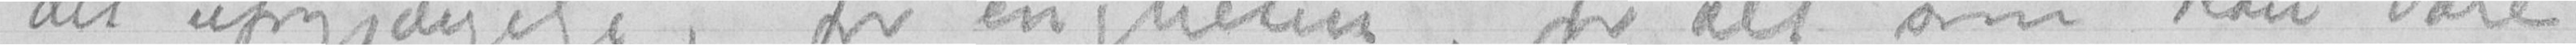det uforgjængelige, for evigheten, for alt, som kan bære
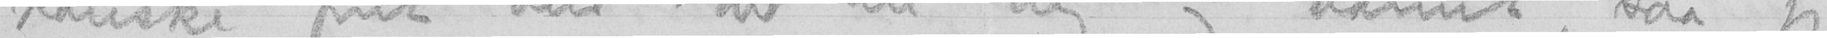kanske fint veir her en dag og varmt, saa jeg
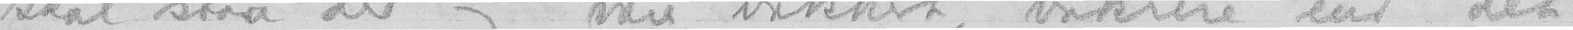skal staa der og være vakkert, vakrere end det
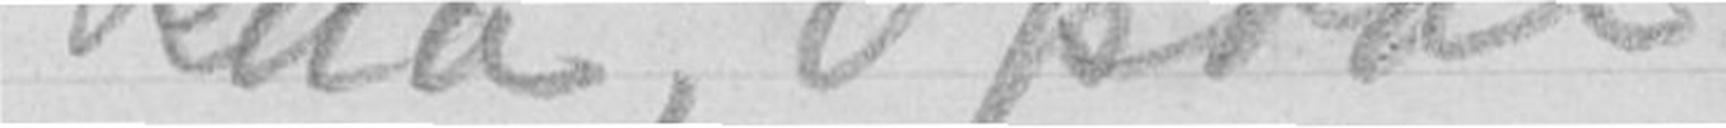Bua, Opdal
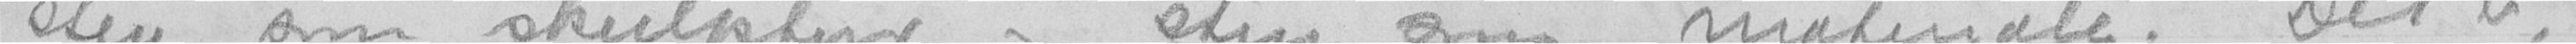sten som skulptur og sten som materiale. Det er,
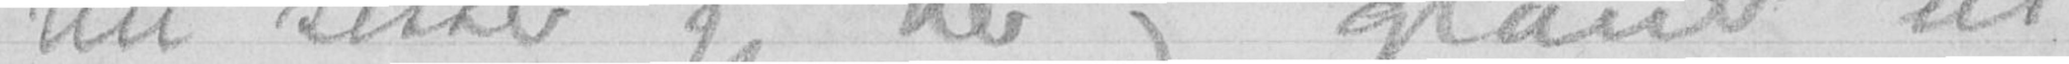nu sitter jeg her og glaner ut
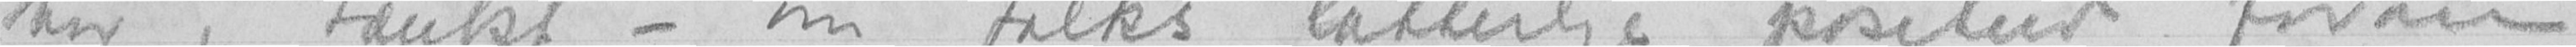har jeg tænkt - om folks latterlige "positur" foran
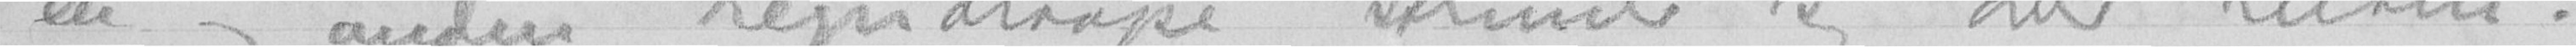en og anden regndraape strimer sig over ruten.
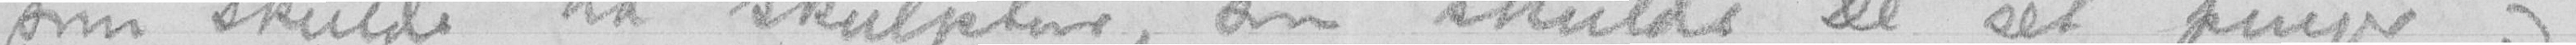som skulde ha skulptur, saa skulde De set penger og
